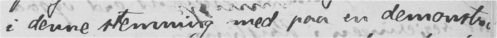i denne stemning med paa en demonstra-
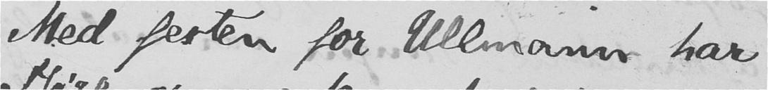Med festen for Ullmann har
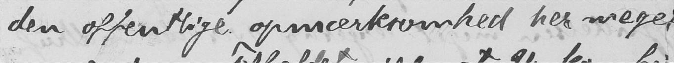den offentlige opmærksomhed her meget
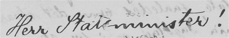Herr Statsminister!
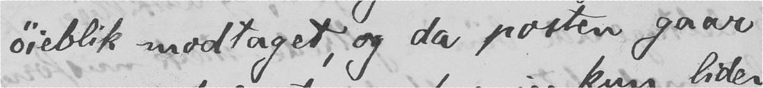øieblik modtaget, og da posten gaar
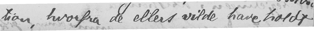tion, hvorfra de ellers vilde have holdt
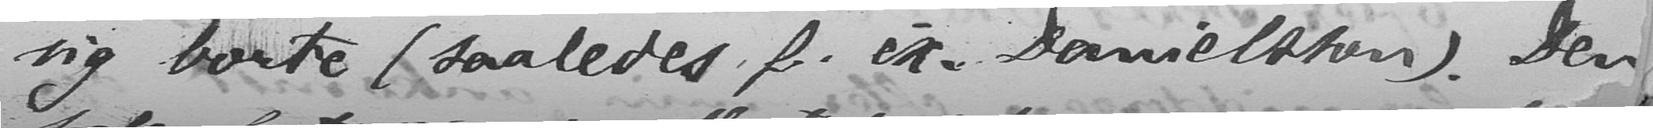sig borte (saaledes f. ex. Danielsson). Den
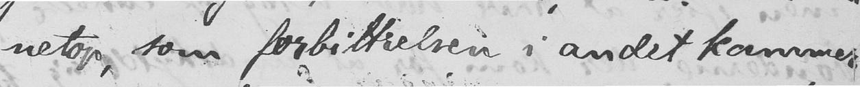netop, som forbittrelsen i andet kammer
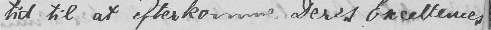tid til at efterkomme Deres Excellences
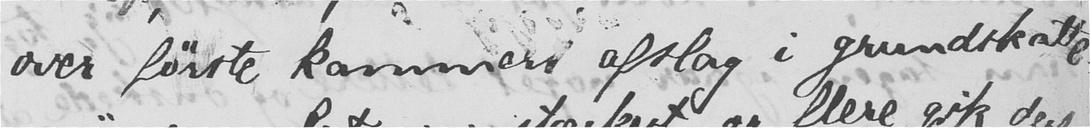over første kammers afslag i grundskatte-
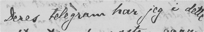Deres telegram har jeg i dette
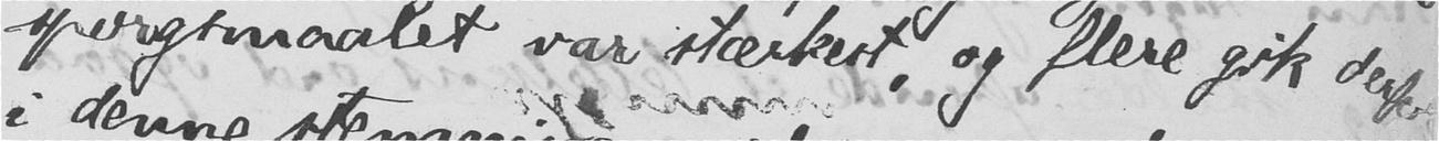spørgsmaalet var stærkest, og flere gik derfor
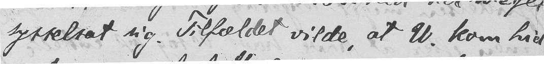sysselsat sig. Tilfældet vilde, at U. kom hid
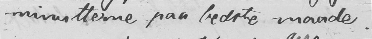minutterne paa bedste maade.
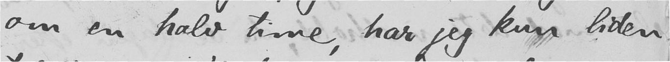om en halv time, har jeg kun liden
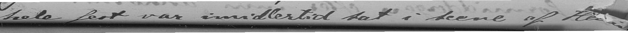hele fest var imidlertid sat i scene af Hedin
